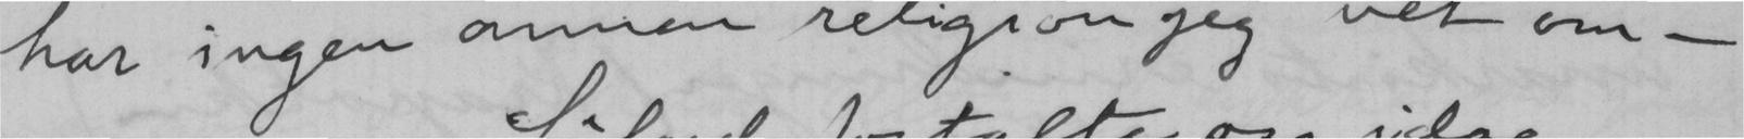har ingen annen religion jeg vet om -
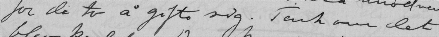for de to å gifte sig. Tenk om det
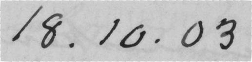18.10.03
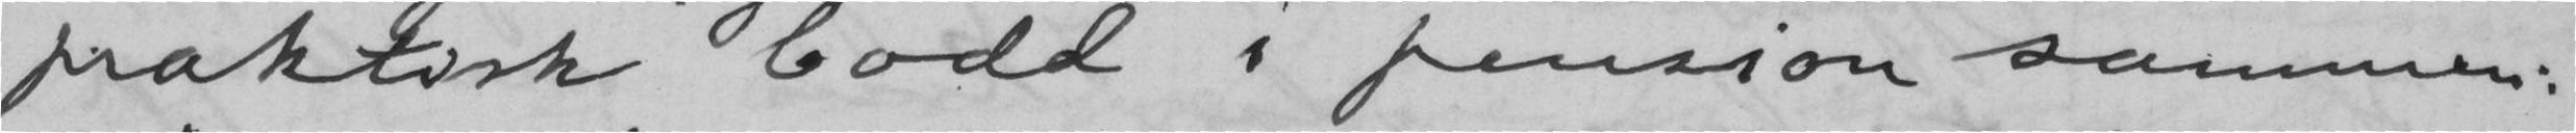paktisk bodd i pension sammen:
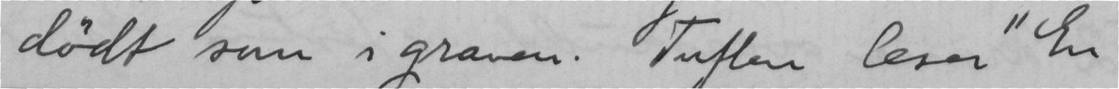dødt som i graven. Tuften leser "En
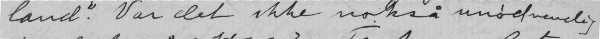land". Var det ikke nokså unødvendig
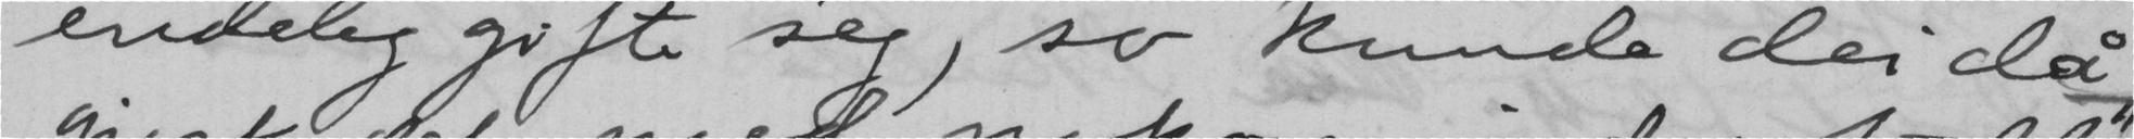endeleg gifte seg, so kunde dei då
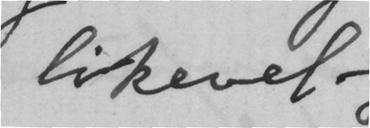likevel-
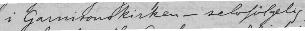i Garnisonskirken - selvfølglig
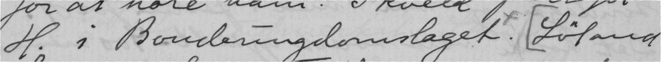H. i Bondeungdomslaget. Løland
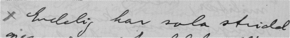x Endelig har sola stridd
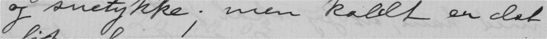og snetukke; men kaldt er det
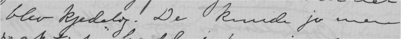blev kjedelig. De kunde jo mere
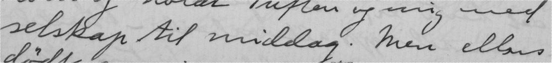selskap til middag. Men ellers
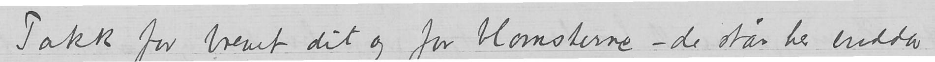Takk for brevet dit og for blomsterne - de står her endda
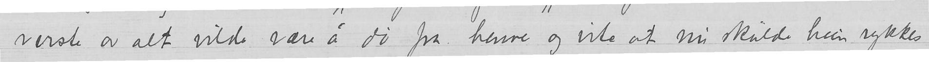verste av alt vilde være å du fra henne og vite at nu skulde hun rykkes
